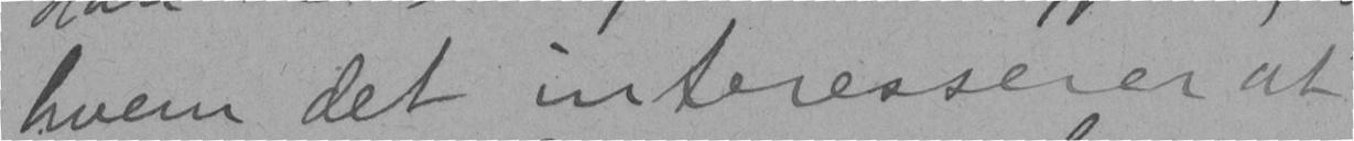hvem det interesserer at
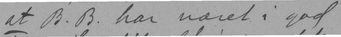at B. B. har været i god
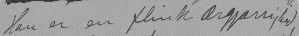Han er en flink Ærgjærrighed,
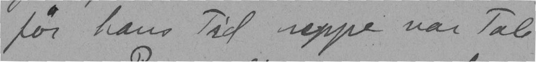før hans Tid neppe var Tale
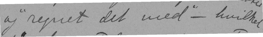og "regnet det med" - hvilket
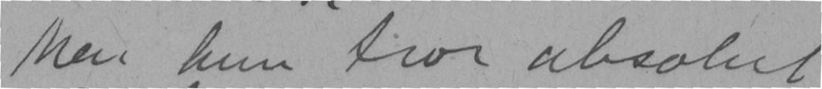Men hun tror absolut
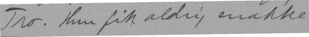Tro. Hun fik aldrig snakke
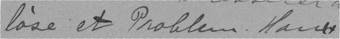løse et Problem. Han
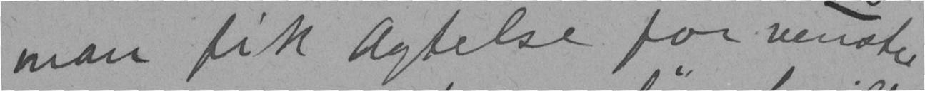man fik agtelse for venstre
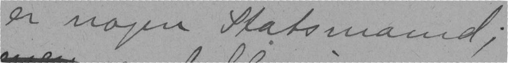er nogen Statsmand;
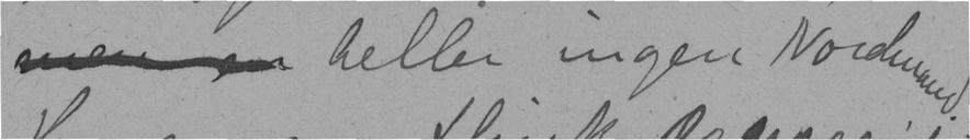heller ingen Nordmand.
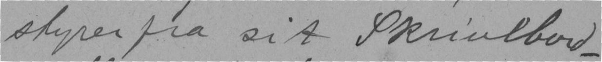styrer fra sit Skrivebord -
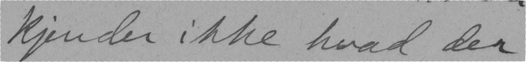kjender ikke hvad der
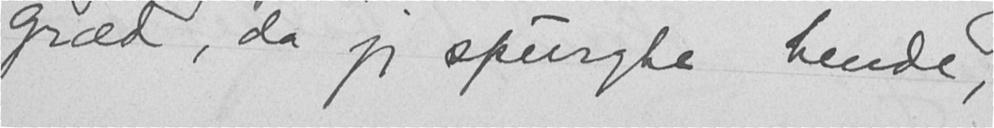græd, da jeg spurgte hende,
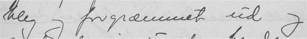bleg og forgræmmet ud og
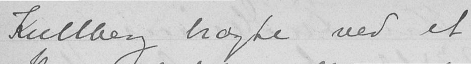Kullberg bragte ved et
